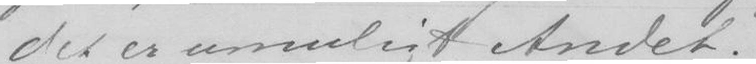det er umuligt Andet.
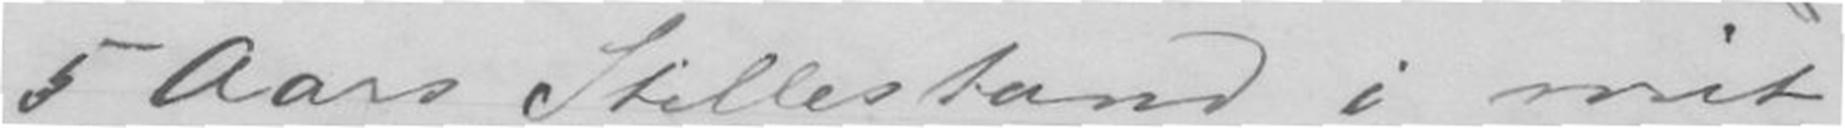5 Aars Stillestand i mit
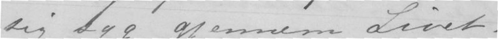sig syg gjennem Livet.
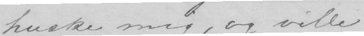huske mig, og ville
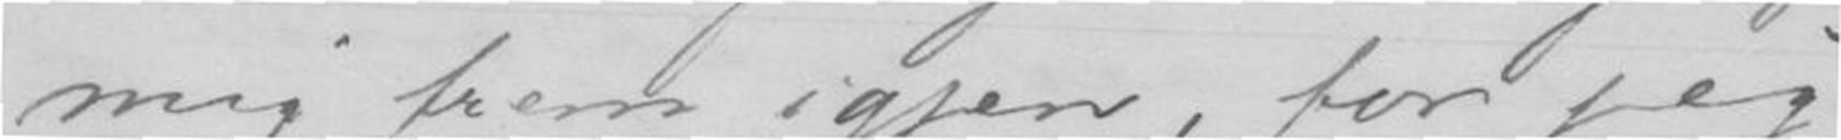mig frem igjen, for jeg
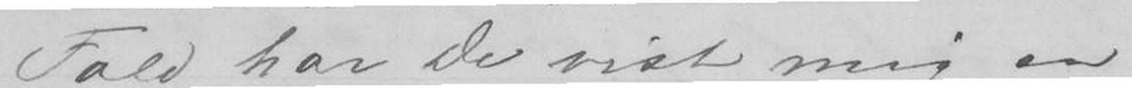Fald har De vist mig en
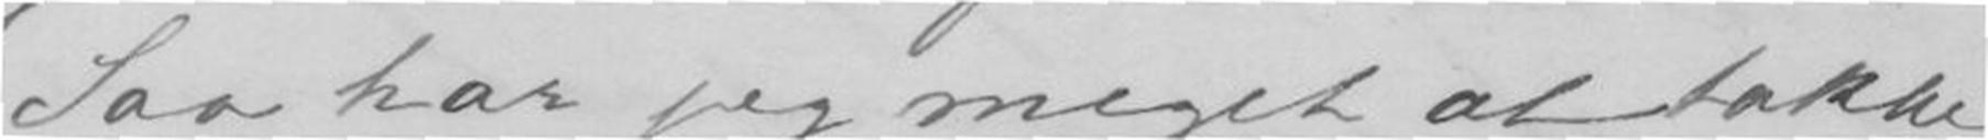Saa har jeg meget at takke
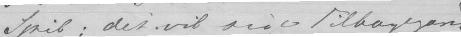Spil; det vil sige Tilbagegang,
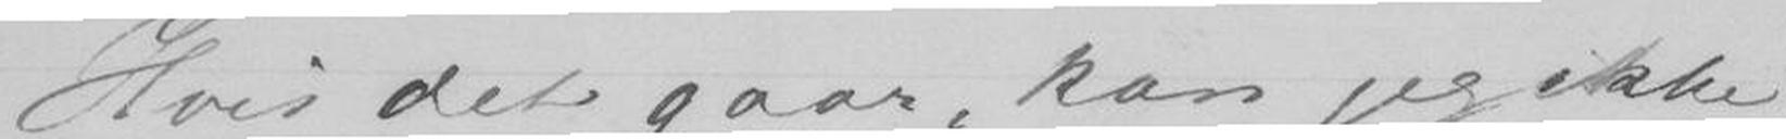Hvis det gaar, kan jeg ikke
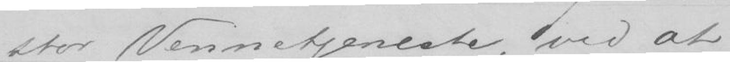stor Vennetjeneste, ved at
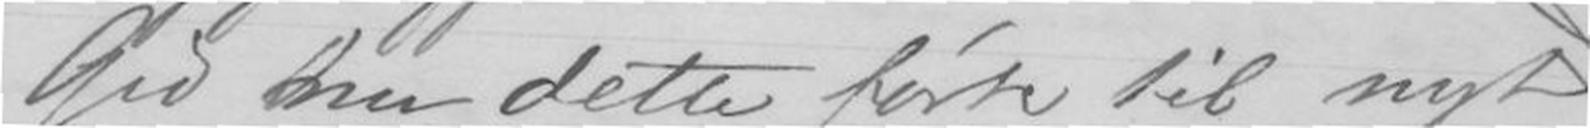Gid nu dette førde til nyt
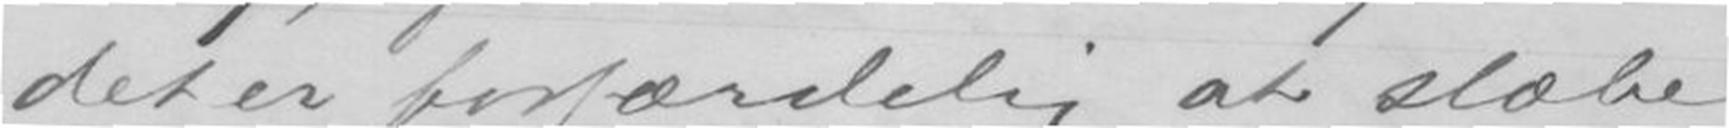det er forfærdelig at slæbe
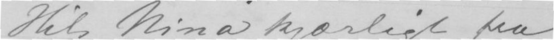Hils Nina kjærligt fra
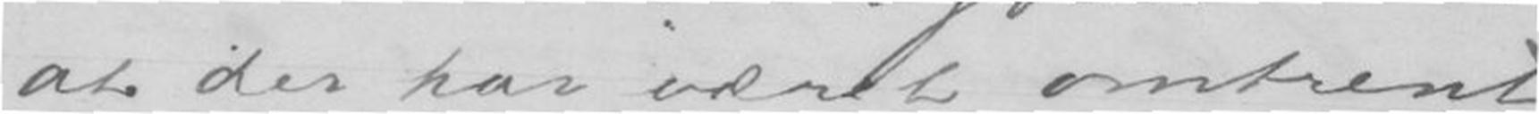at der har været omtrent
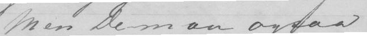Men De maa ogsaa
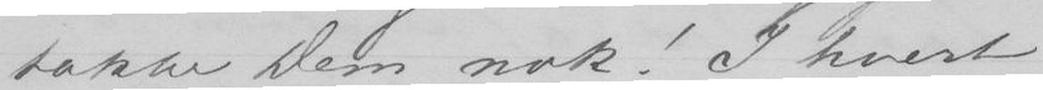takke Dem nok! I hvert
